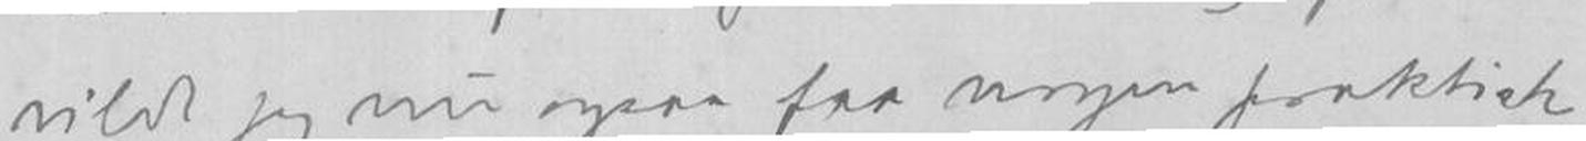vilde jeg nu ogsaa faa nogen praktisk
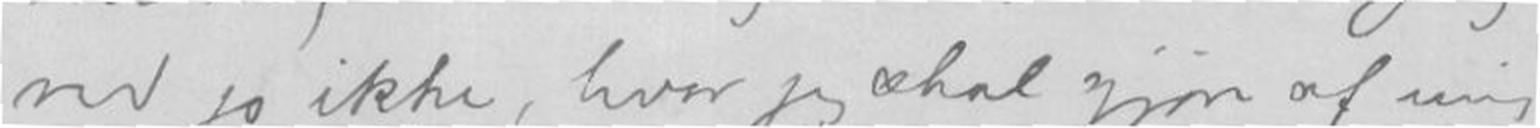ved jo ikke, hvor jeg skal gjøre af mig
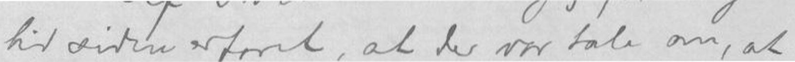tid siden erfaret, at der var tale om, at
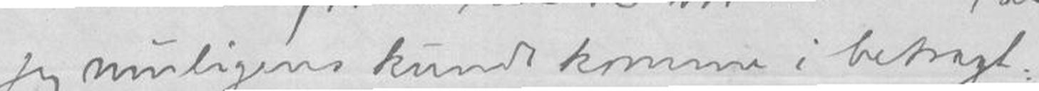jeg muligens kunde komme i betragt-
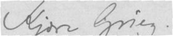Kjære Grieg!
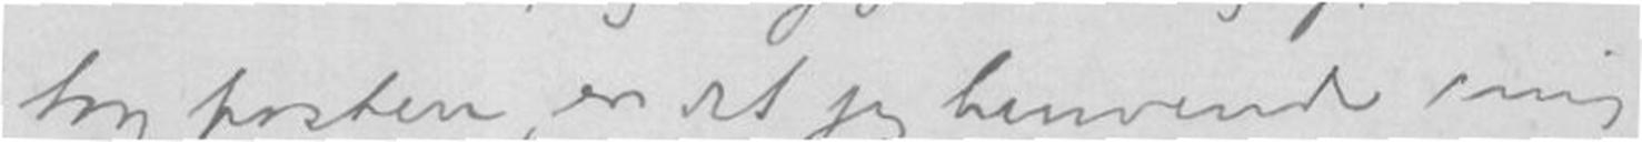tog posten, er det jeg henvender mig
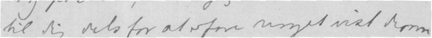til dig dels for at høre noget vist derom
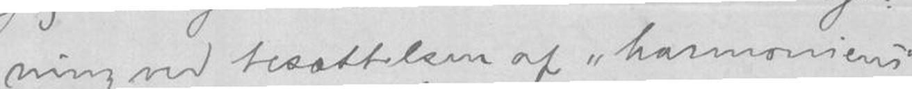ning ved besættelsen af "harmoniens"
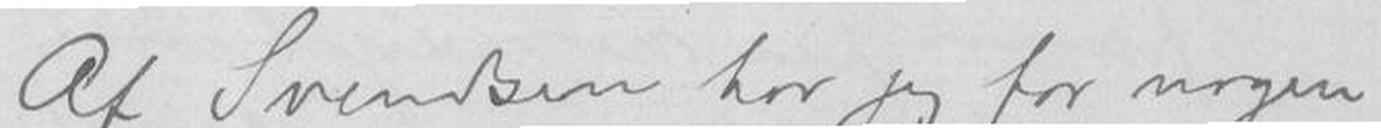Af Svendsen har jeg for nogen
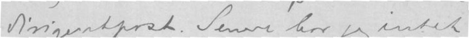dirigentpost. Senere har jeg intet
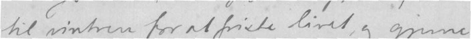til vinteren for at friste, livet og gjerne
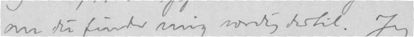im De finder mig værdig dertil. Jeg
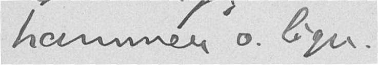hammer o. lign.
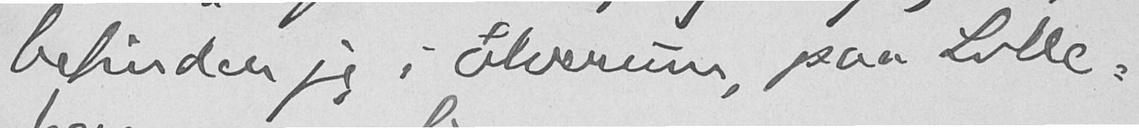befinder jeg i Elverum, paa Lille-
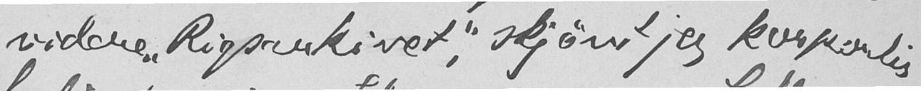videre "Rigsarkivet", skjønt jeg korporlig
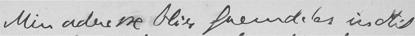Min adresse blir fremdeles indtil
